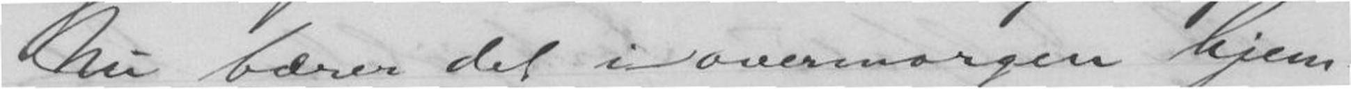Nu bærer det i overmorgen hjem-
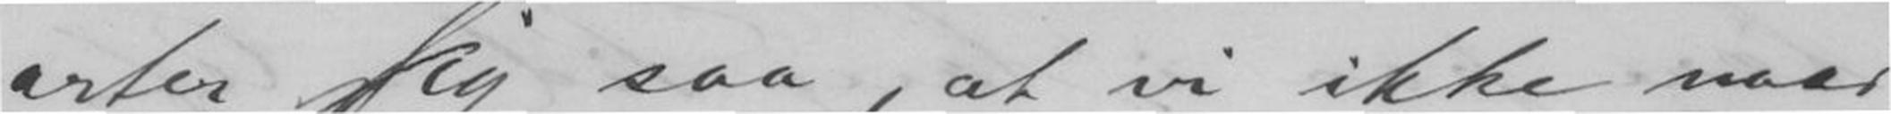arter sig saa, at vi ikke naar
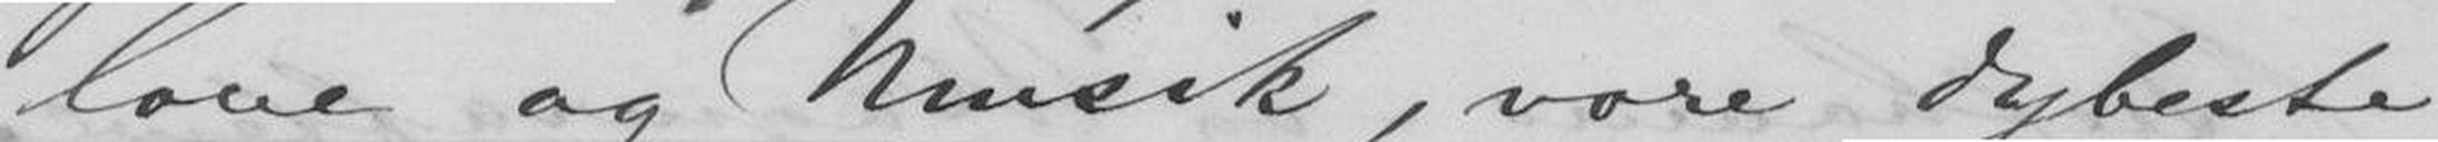love og Musik, vore dybeste
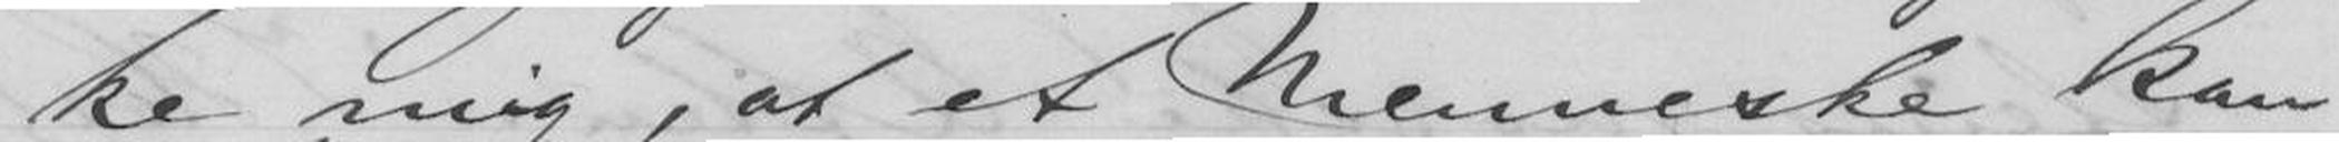ke mig, at et Menneske kan
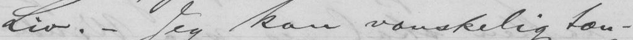Liv. - Jeg kan vanskelig tæn-
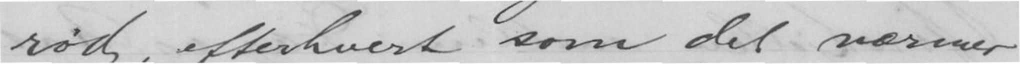rød, efterhvert som det nærmer
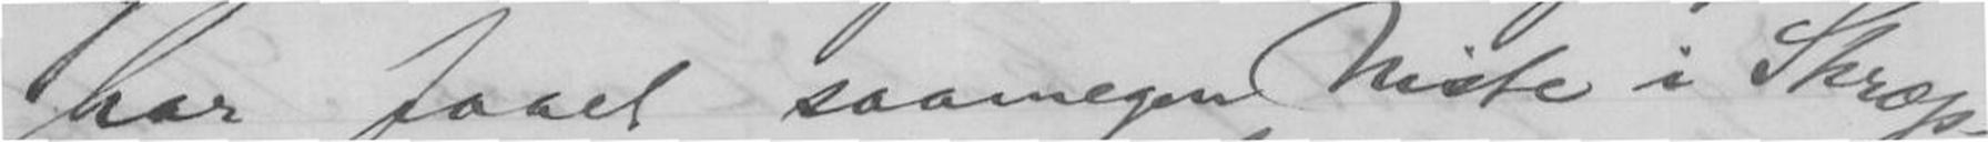har faaet saameget Niste i Skræp-
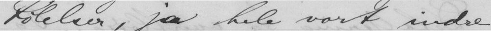Følelser, ja hele vort indre
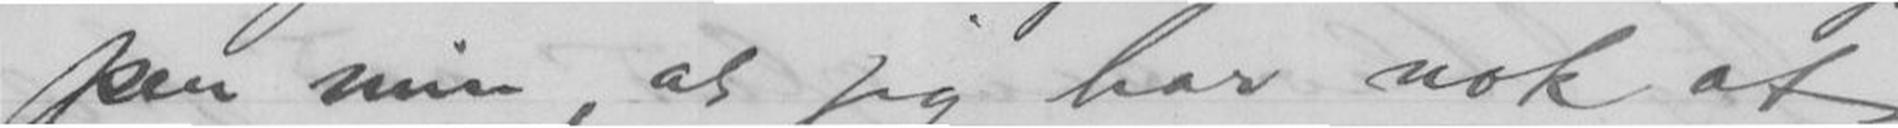pen min, at jeg har nok at
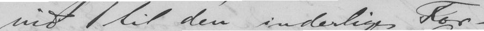ind til den inderlige For-
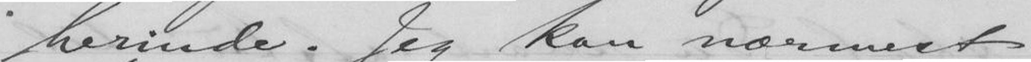herinde. Jeg kan nærmest
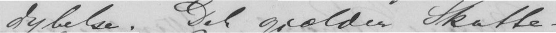dybelse. Det gjælder Skatte-
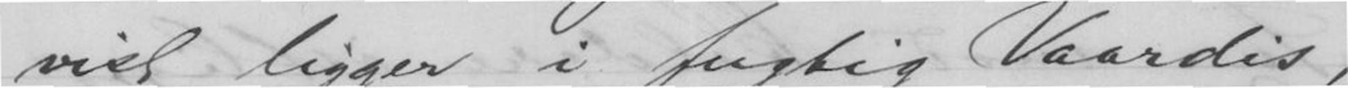vist ligger i fugtig Vaardis,
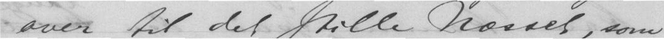over til det stille Næsset, som
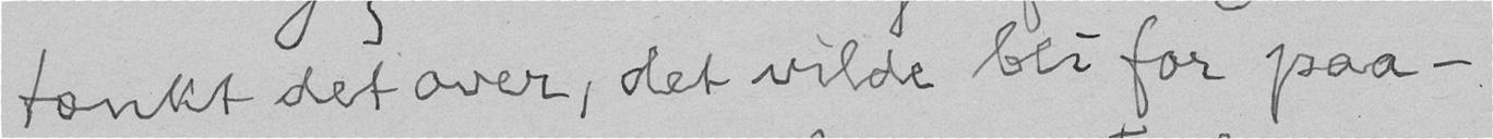tænkt det over, det vilde bli for paa-
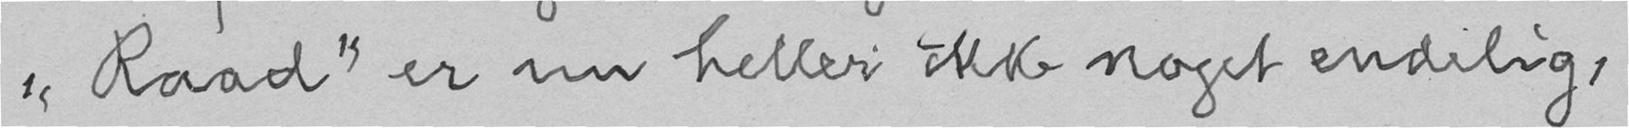"Raad" er nu heller ikke noget endelig,
